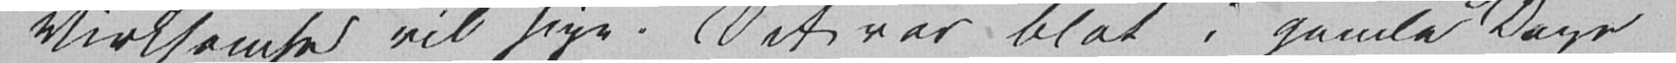Virksomhed vil sige. Det var blot i gamle Dage
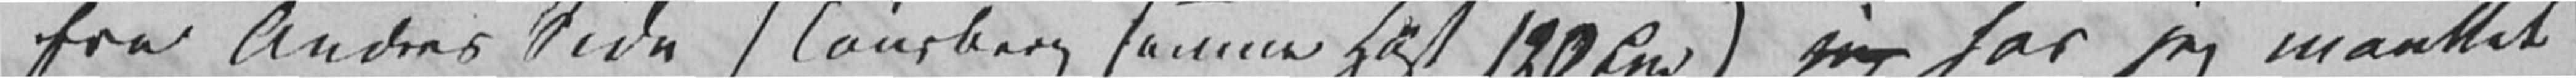fra Andres Side (Tønsberg samme Høst 120 Spd) jeg har jeg maattet
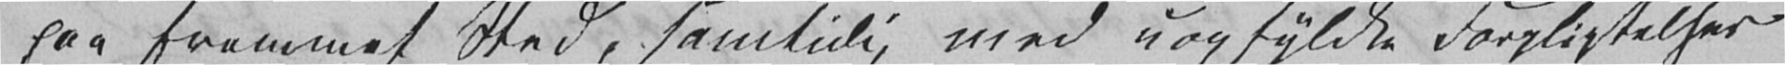paa fremmet Sted, samtidig med uopfyldte Forpligtelser
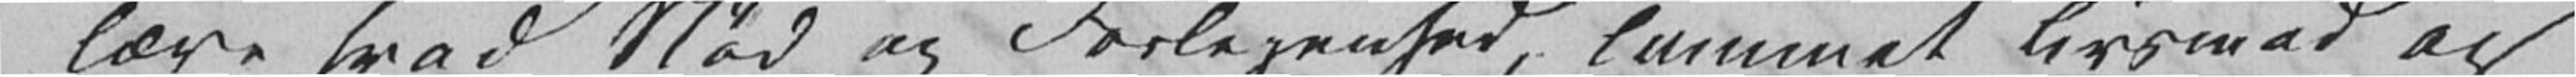lære hvad Nød og Forlegenhed, lammet Livsmod og
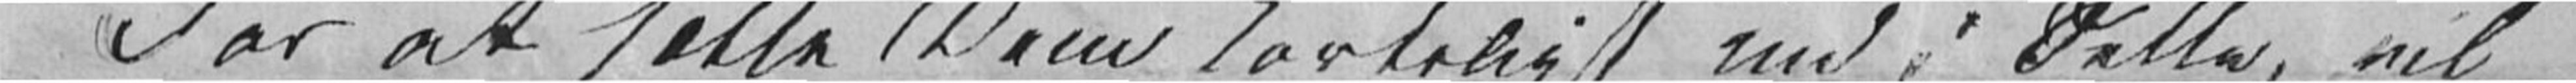For at sætte Dem korteligt ind i dette, vil
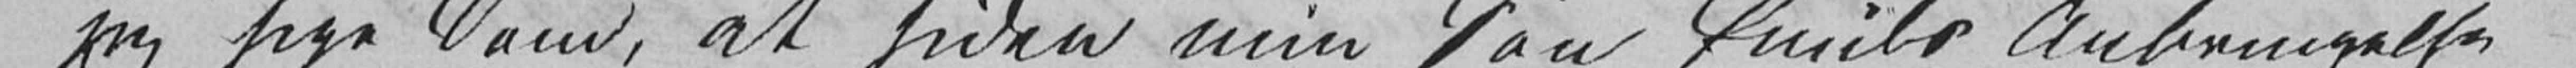jeg sige Dem, at siden min Søn Emils Anbringelse
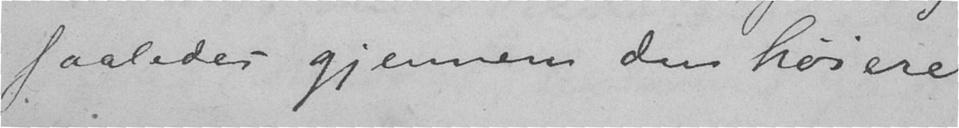saaledes gjennem den høiere
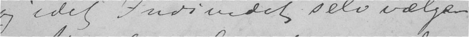og idet Individet selv vælger
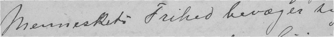Menneskets Frihed bevæger sig
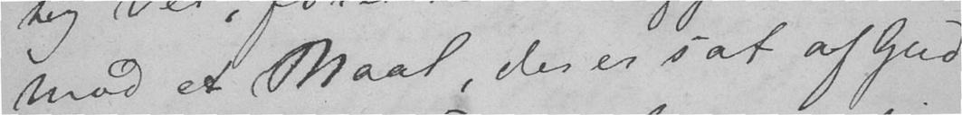mod et Maal, der er sat af Gud
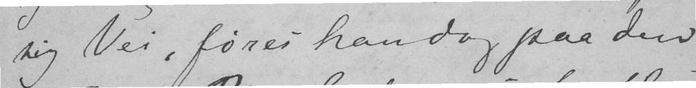sig Vei, føres han dog paa den
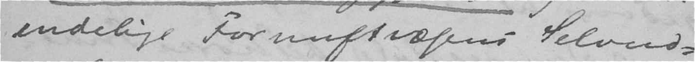endelige Fornuftvæsens Selvud-
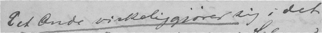Det Onde virkeliggjører sig i det
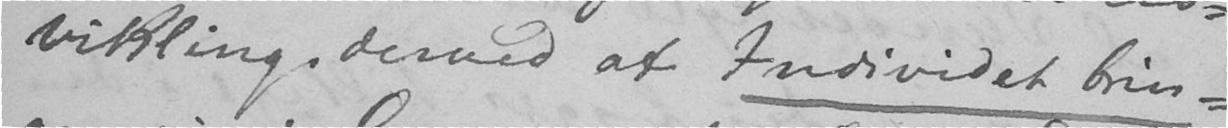vikling derved at Individet brin-
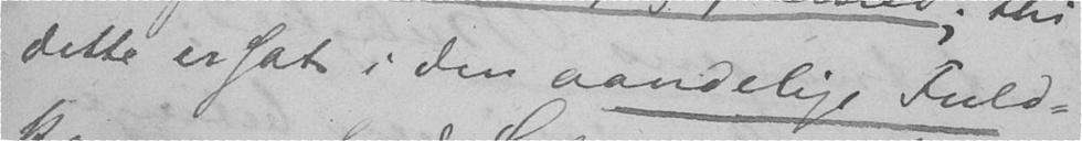dette er sat i den aandelige Fuld-
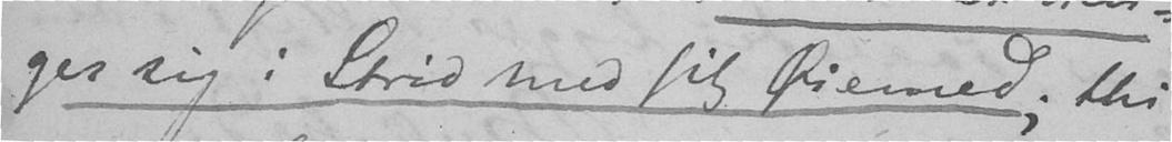ger sig i Strid med sit Øiemed; thi
